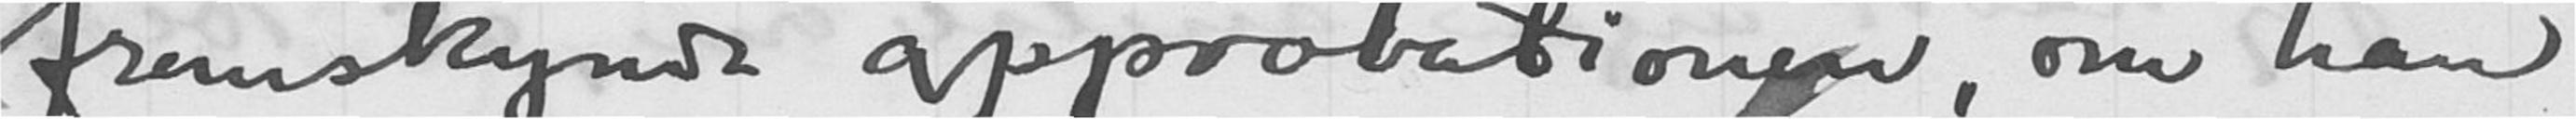fremskynde approbationen, om han
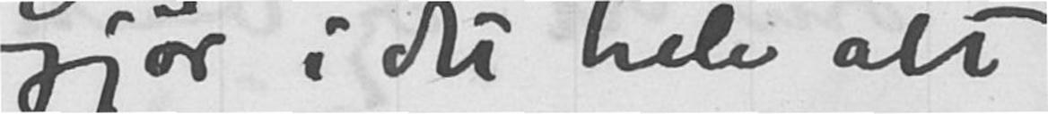Gjør i det hele alt
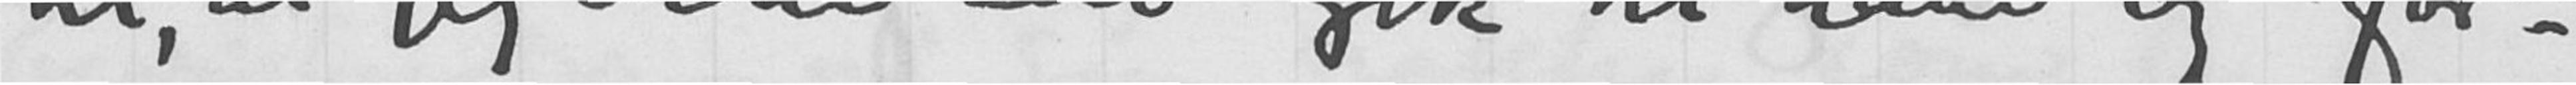til, at jeg ikke selv gik til ham og for-
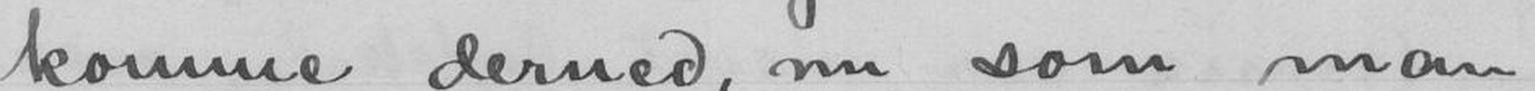komme derned, nu som man
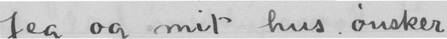Jeg og mit hus ønsker
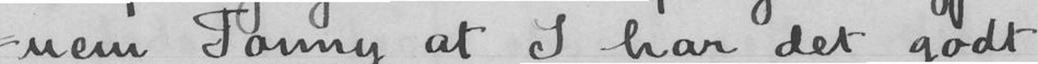nem Fanny at I har det godt
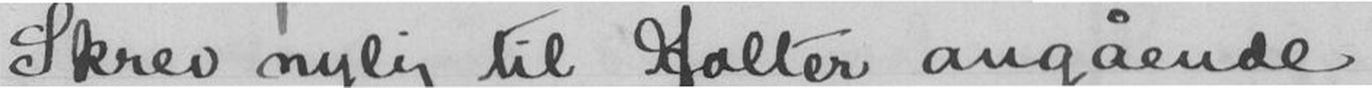Skrev nylig til Holter angående
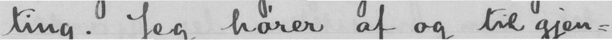ting. Jeg hører af og til gjen-
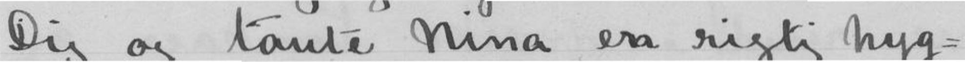Dig og tante Nina en rigtig hyg-
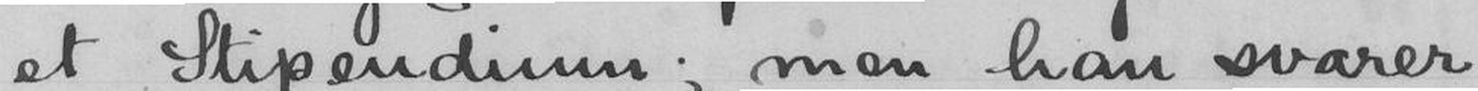et Stipendium; men han svarer
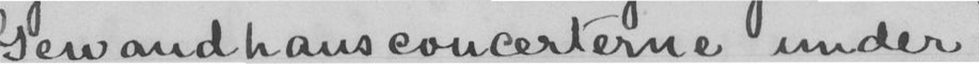Gewandhausconcerterne under
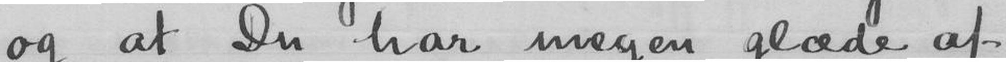og at Du har megen glæde af
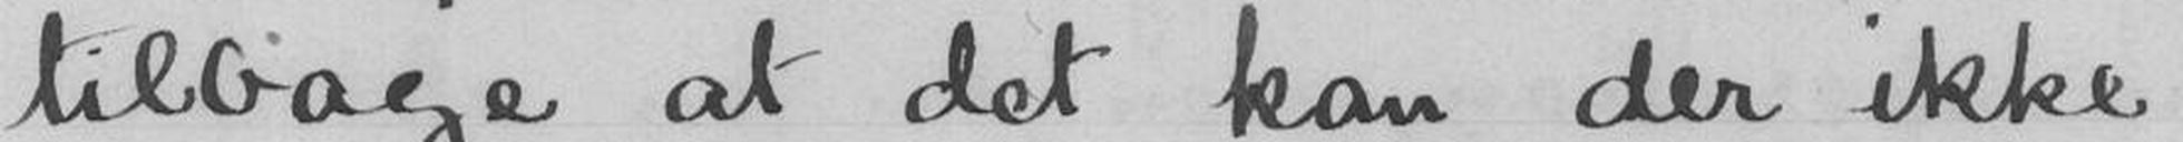tilbage at det kan der ikke
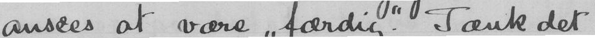ansees at være færdig. Tænk det
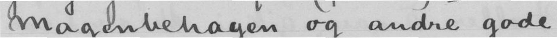Magenbehagen og andre gode
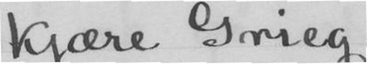Kjære Grieg
